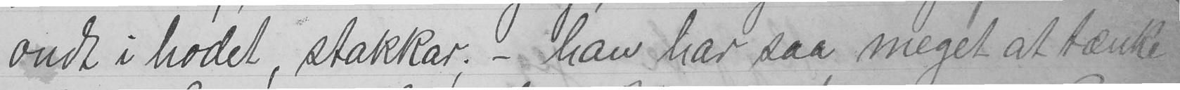ondt i hodet, stakkar; - han har saa meget at tænke
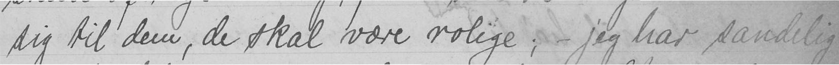sig til dem, de skal være rolige; - jeg har sandelig
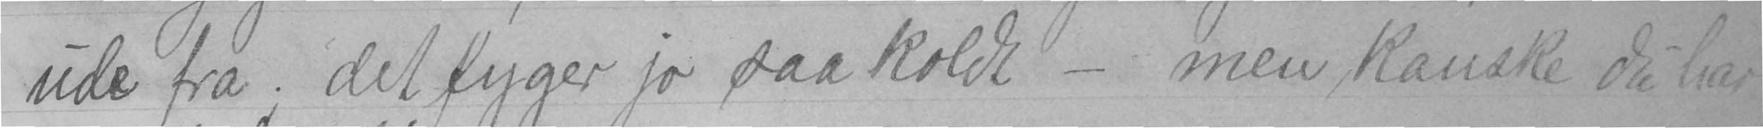ude fra; det fyger jo saa koldt - men kanske du har
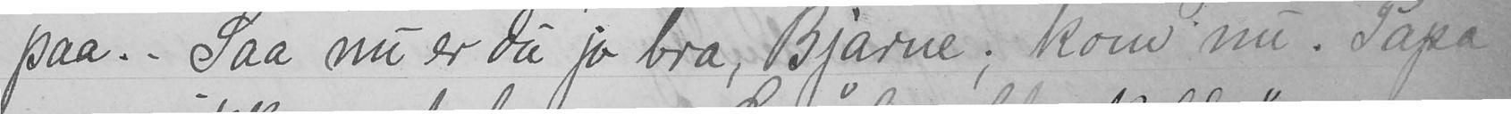paa . . Saa nu er du jo bra, Bjarne; kom nu. Papa
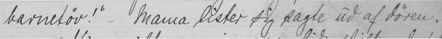barnetøv!" - Mama lister sig sagte ud af døren.
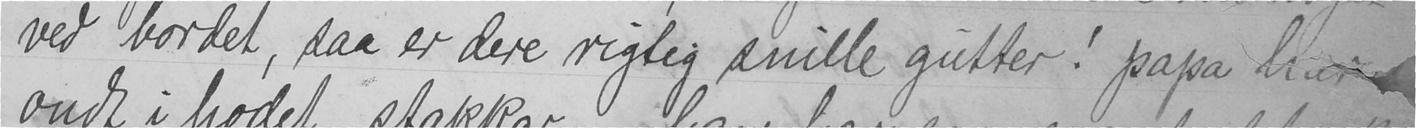ved bordet, saa er dere rigtig snille gutter! papa har
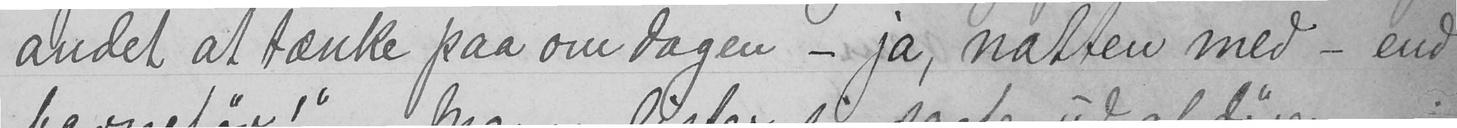andet at tænke paa om dagen - ja, natten med - end
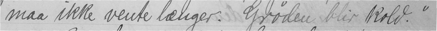maa ikke vente længer. Grøden blir kold."
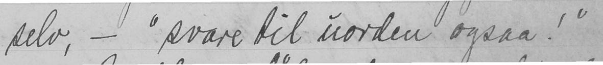selv, - "svare til uorden ogsaa!"
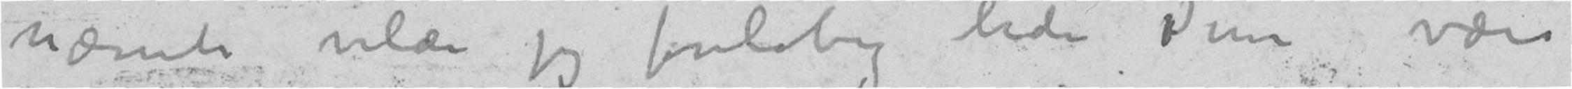nævnte vilde jeg foreløbig bede Dem være
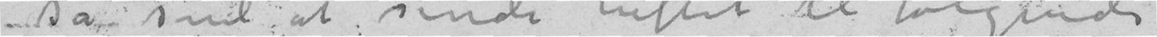så snil at sende heftet til følgende
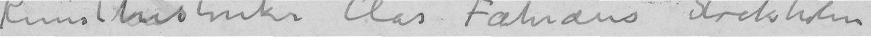Kunsthistoriker Clas Fähræus Stockholm
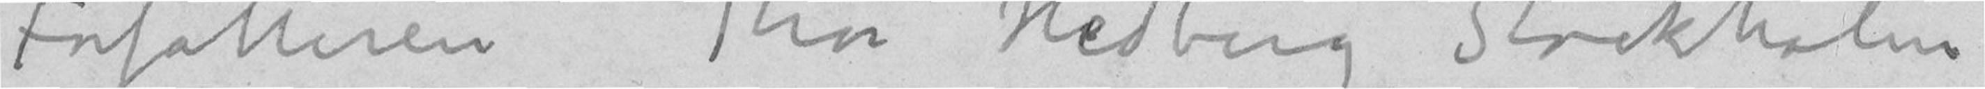Forfatter Thor Hedberg Stockholm
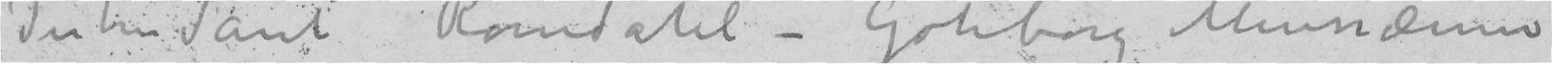Intendant Romdahl – Göteborg Mussæum
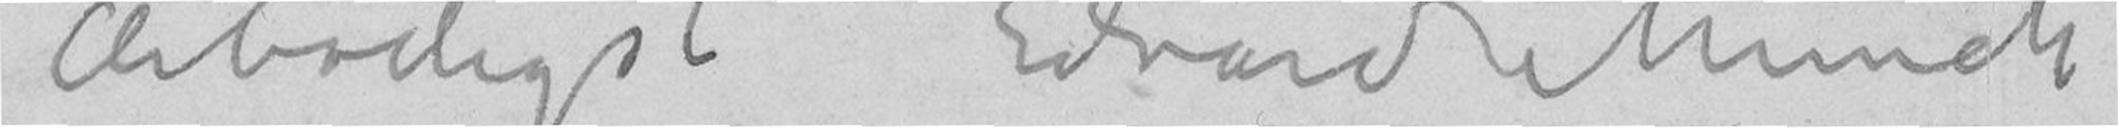Ærbødigst Edvard Munch
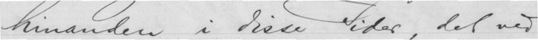hinanden i disse Tider, det ved
